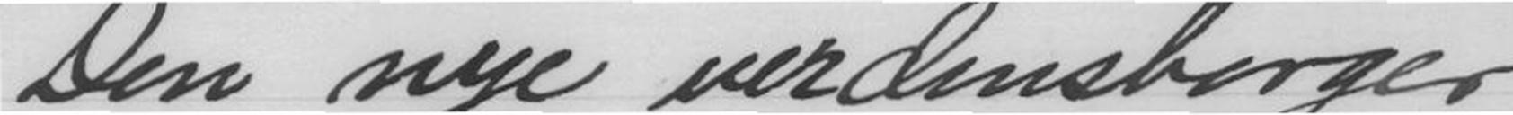Den nye verdensborger
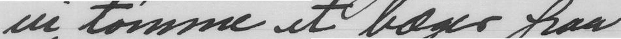vi tømme et bæger paa
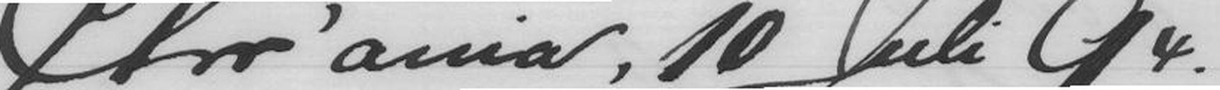Chr'ania, 10 Juli 94.
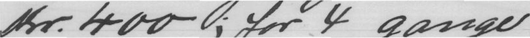Kr. 400; for 4 gange
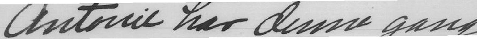Antonie har denne gang
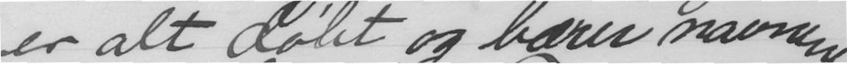er alt døbt og bærer navnene
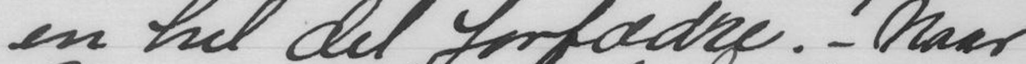en hel del forfædre. - Naar
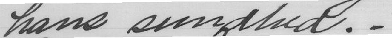hans sundhed. -
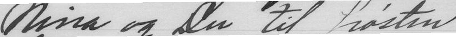Nina og Du til høsten
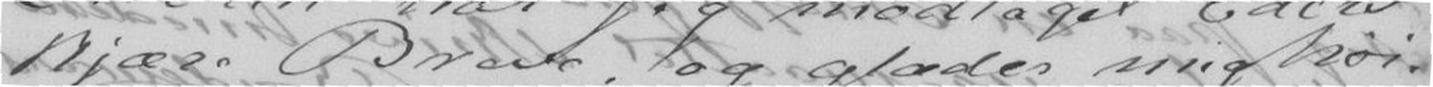kjære Breve, og glæder mig høi-
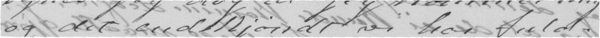og det endskjøndt vi har fuld-
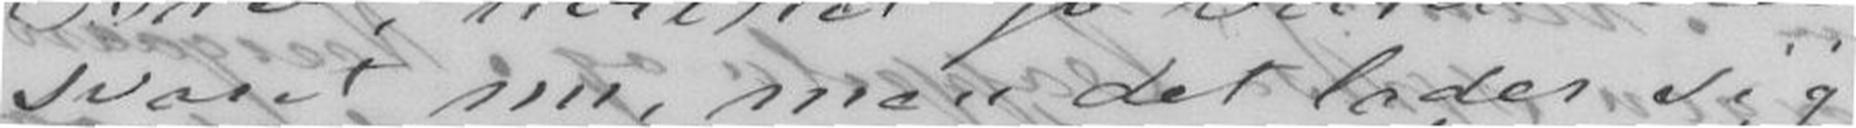svaret nu, men det lader sig al
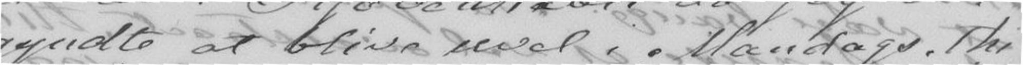gyndte at blive uvel i Mandags, thi
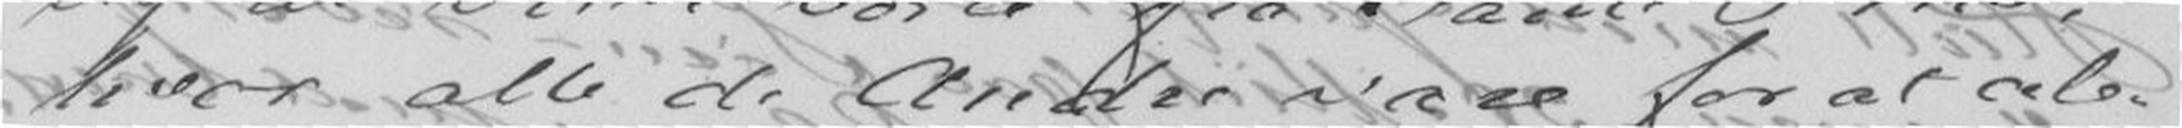hvor alle de Ander vare for at ab-
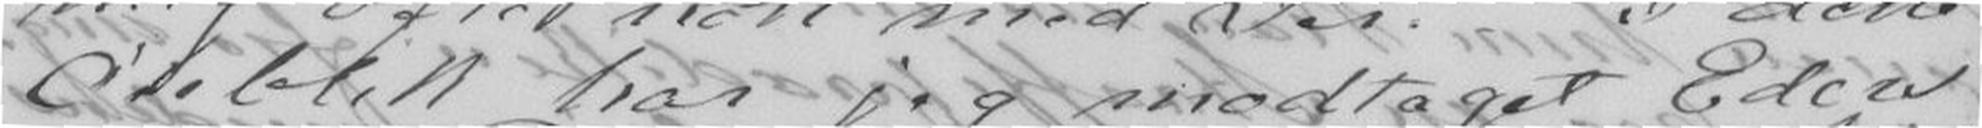Øieblik har jeg modtaget Eders
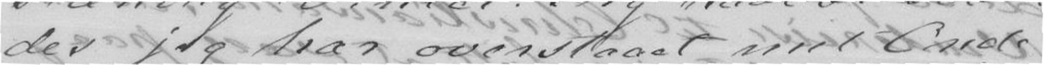es jeg har overstaaet mit Onde
